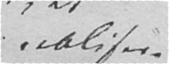realisere
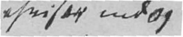afviser endog
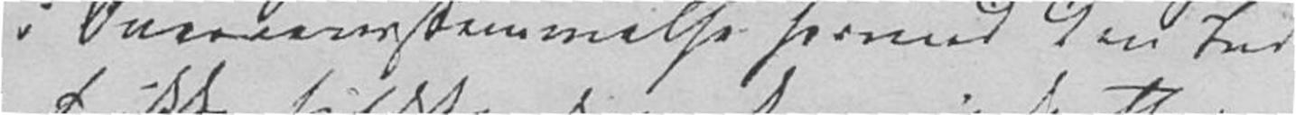i Overeensstemmelse hermed den Ind-
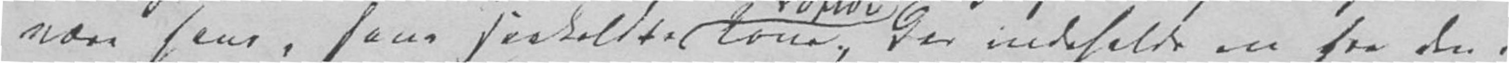være hans, hans saakaldte Love, der indeholde en fra den i
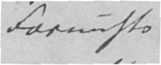Fornufts
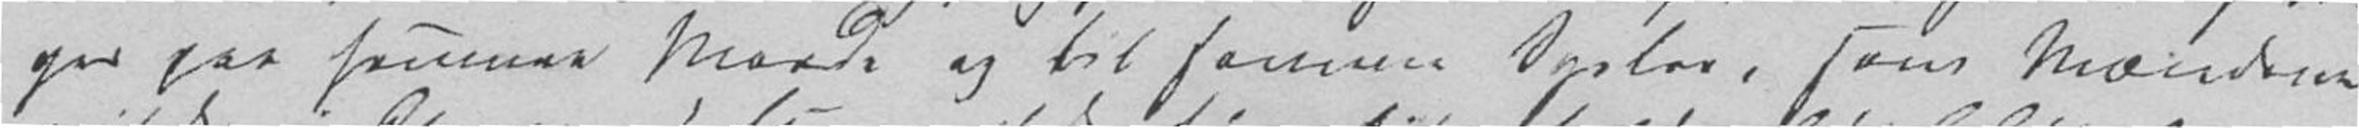ges paa samme Maade og til samme Sysler, som Mænderne
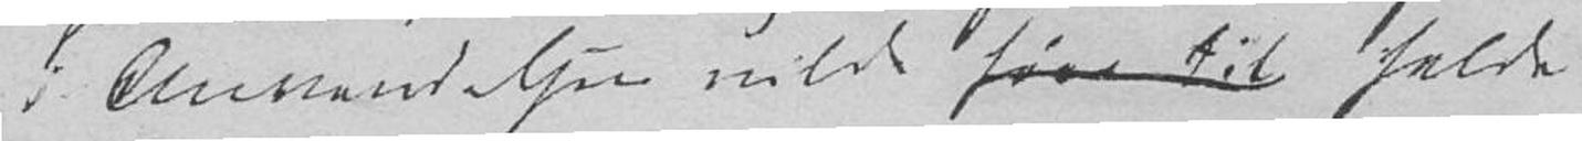i Anvendelsen vilde føre til falde
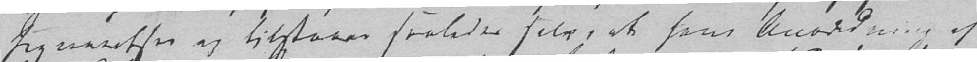seqventser og tilstaaer saaledes selv, at hans Anordning af
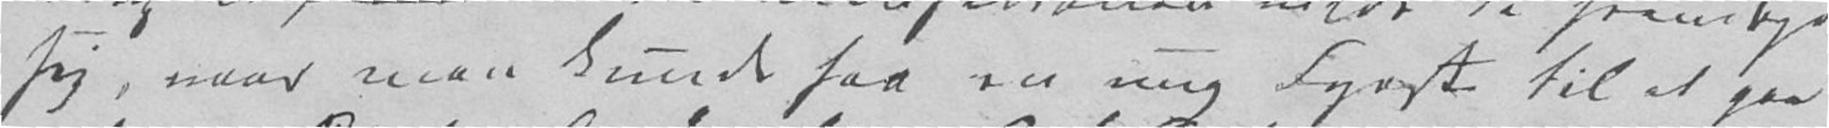sig, naar man kunde faa en ung Fyrste til at gaa
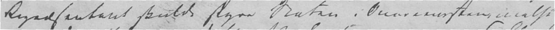Repræsentant skulde styre Staten i Overeensstemmelse
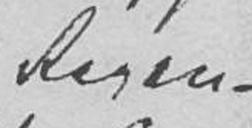Regen-
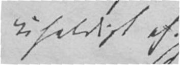uheldigt af.
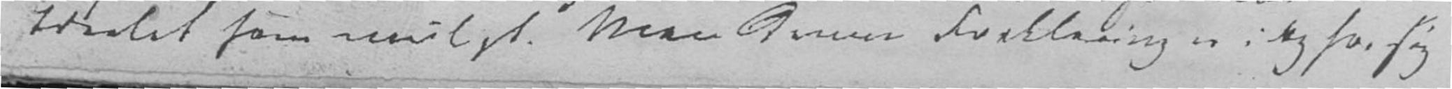Idealet som muligt. Men denne Forklaring er i og for sig
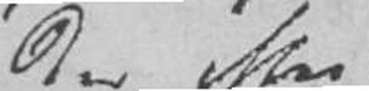der efter
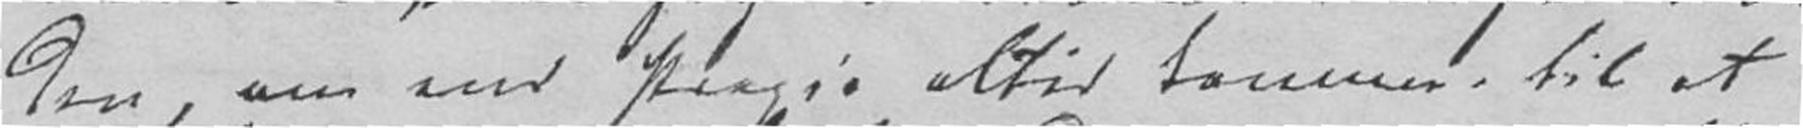den, om end Praxis altid kommer til at
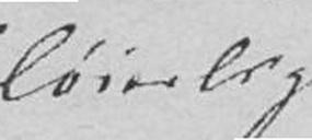løierlig
